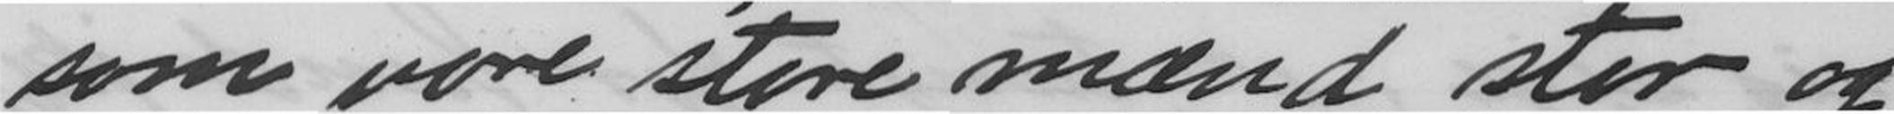som vore store mænd stor og
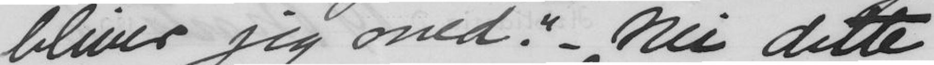bliver jeg med." - Nei dette
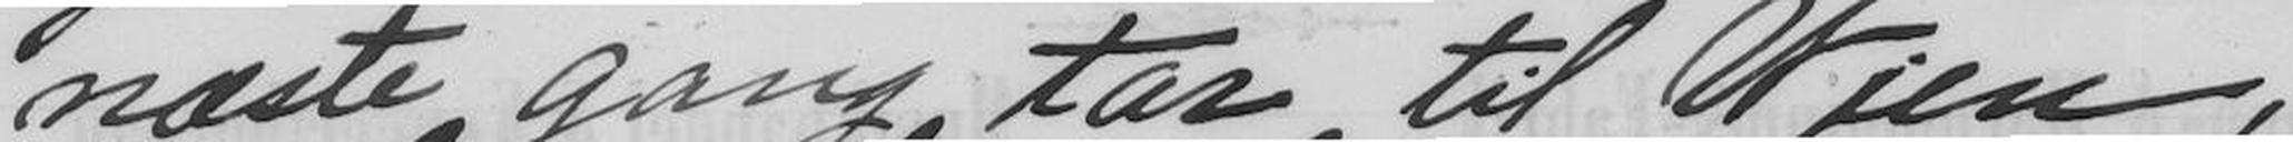næste gang tar til Wien,
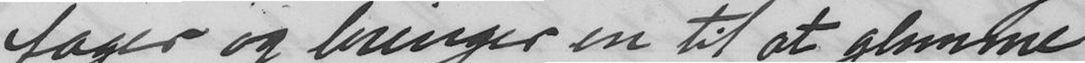fager og bringer en til at glemme
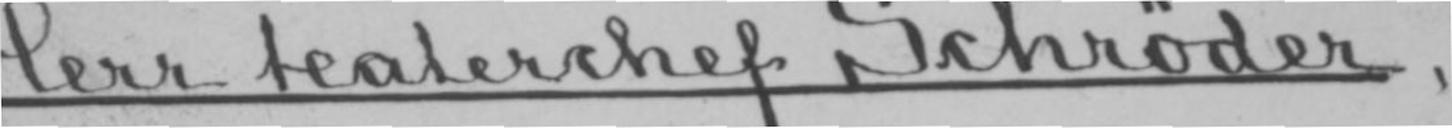Herr teaterchef Schrøder,
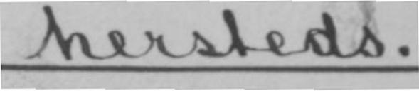hersteds.
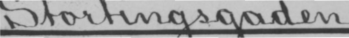Stortingsgaden
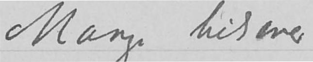Mange hilsener
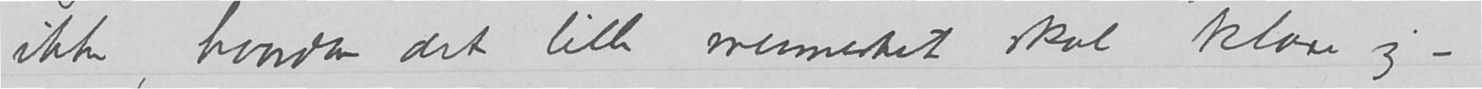ikke, hvordan det lille mennesket skal klare sig –-.
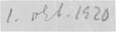1 okt 1920
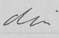din
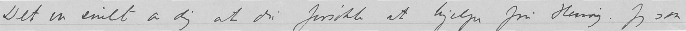Det varer snilt av dig at du forsøkte at hjelpe fru Hennig. Jeg saa
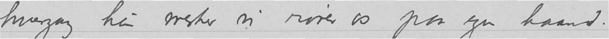hvergang hun merker vi rører os paa egen haand.
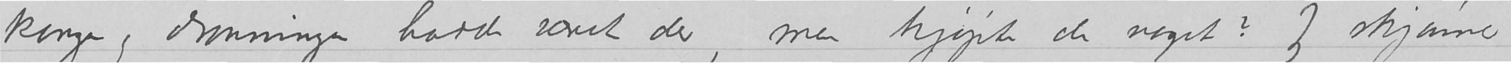kongen og dronningen hadde været der, men kjøpte de noget? Jeg skjønner
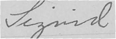Sigrid
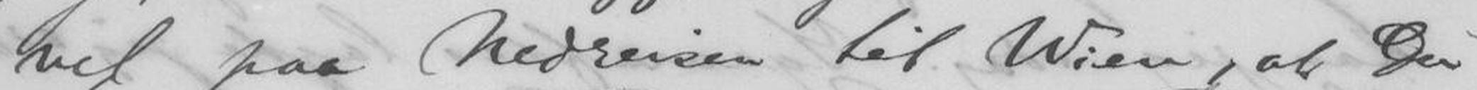vel paa Nedreisen til Wien, at Du
